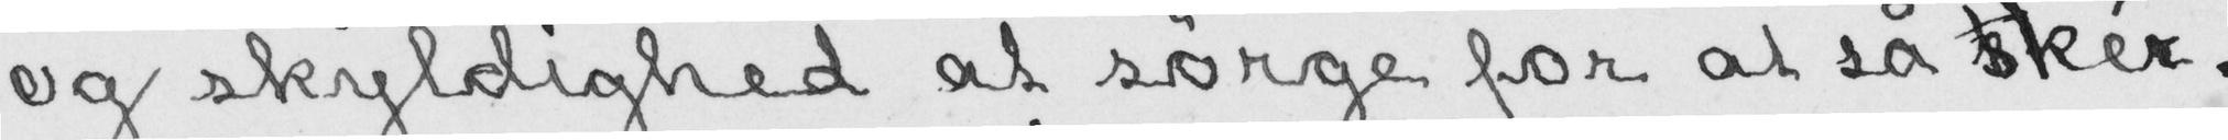og skyldighed at sørge for at så skér.
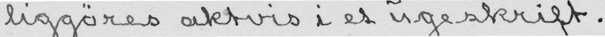liggøres aktvis i et ugeskrift.
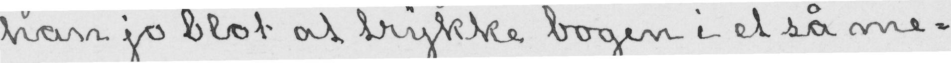han jo blot at trykke bogen i et så me-
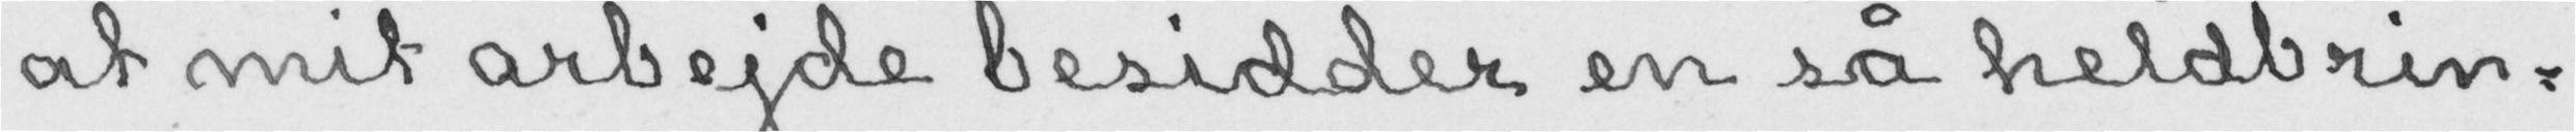at mit arbejde besidder en så heldbrin-
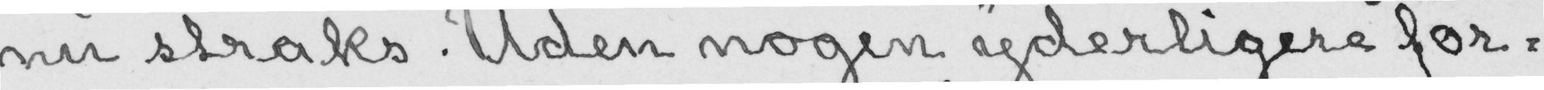nu straks. Uden nogen yderligere for-
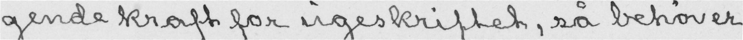gende kraft for ugeskriftet, så behøver
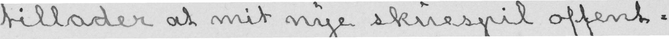tillader at mit nye skuespil offent-
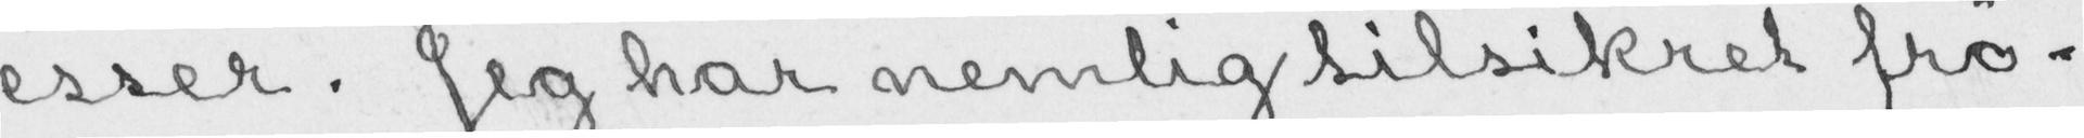esser. Jeg har nemlig tilsikret frø-
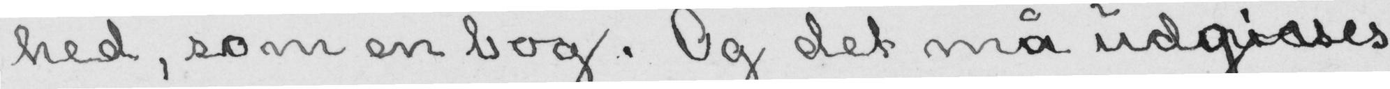hed, som en bog. Og det må udgives
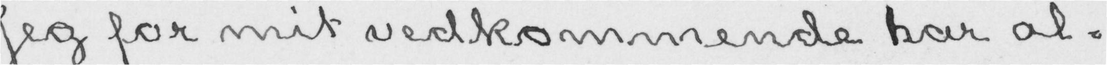Jeg for mit vedkommende har al-
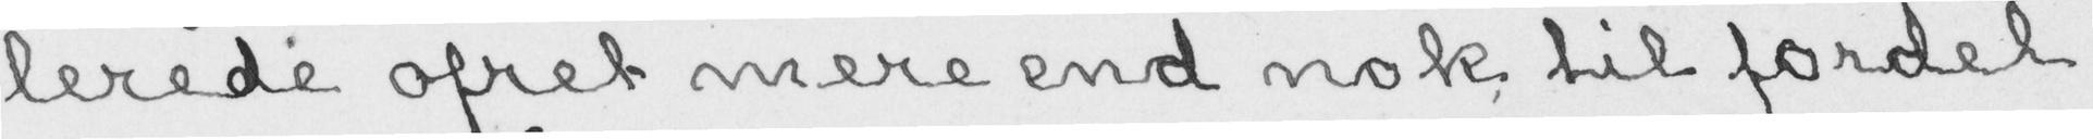lerede ofret mere end nok til fordel
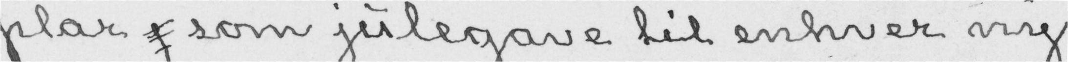plar  som julegave til enhver ny
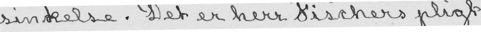sinkelse. Det er herr Fischers pligt
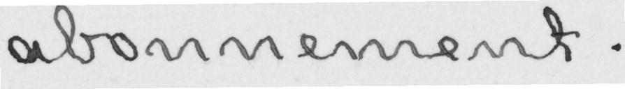abonnement.
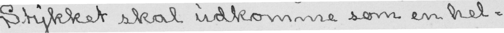Stykket skal udkomme som en hel-
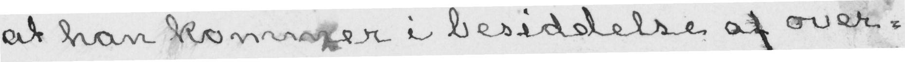at han kommer i besiddelse af over-
